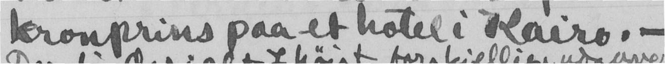kronprins paa et hotel i Kairo. -
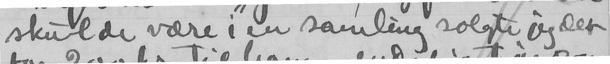skurde være i en samling solgte jeg det
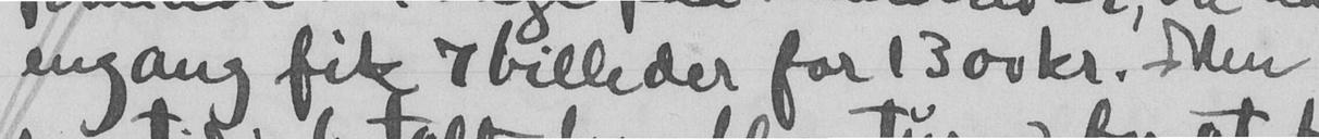engang fik 7 billeder for 1300 kr. Men
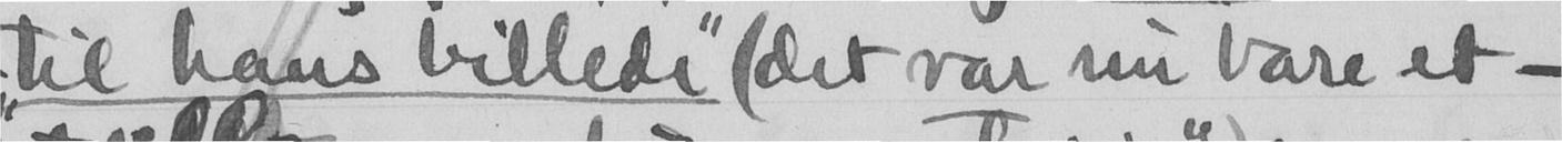"til hans billede" (det var nu bare et -
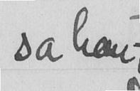sa han
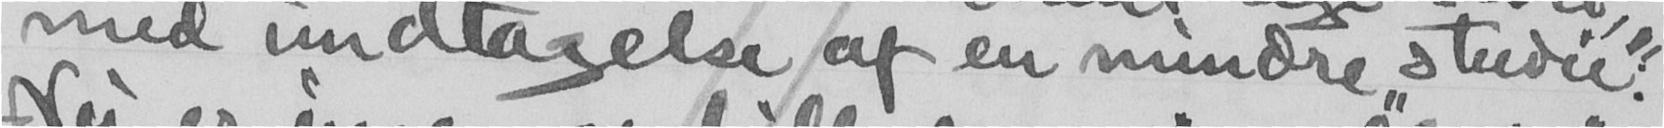med undtagelse af en mindre studie;
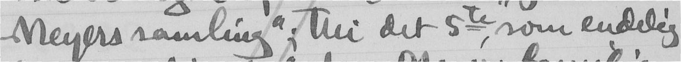"Meyers samling"; thi det 5te, som endelig
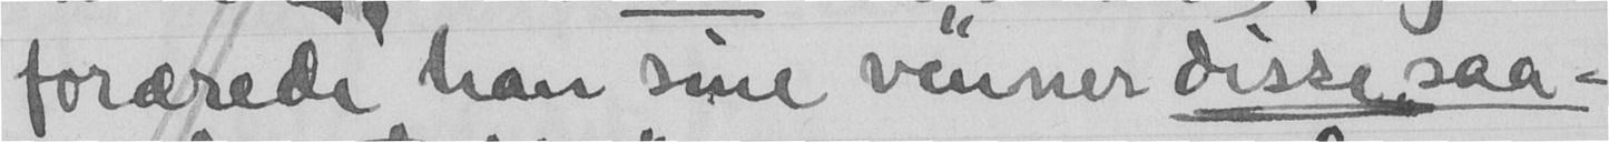forærede han sine venner disse saa-
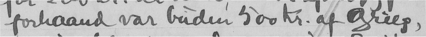forhaand var buden 500 kr. af Grieg,
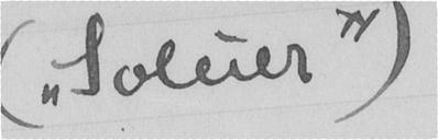"Soleier")
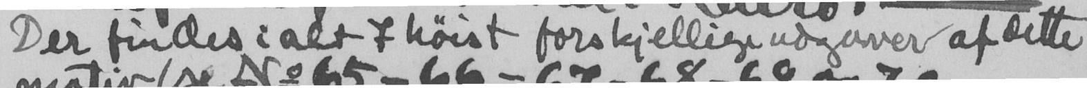Der findes i alt 7 høist forskjellige udgaver af dette
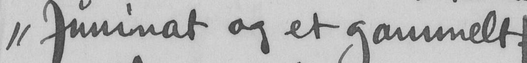"Juinat og et gammelt
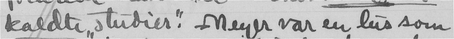kaldte "studier". Meyer var en lus som
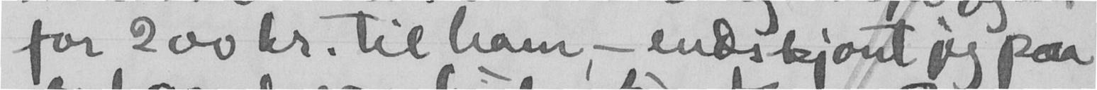for 200 kr. til ham - endskjønt jeg paa
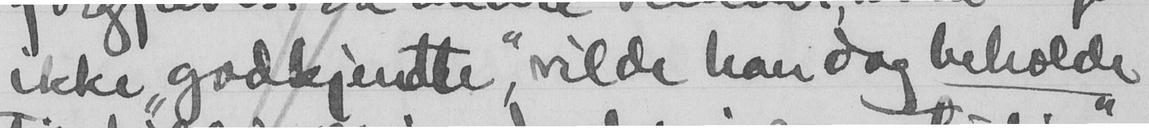ikke "godkjendte" vilde han dog beholde
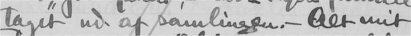taget ud af samlingen. - Alt mit
